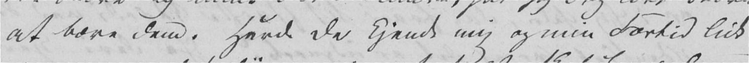at bære dem. Havde De kjendt mig og min Fortid lidt
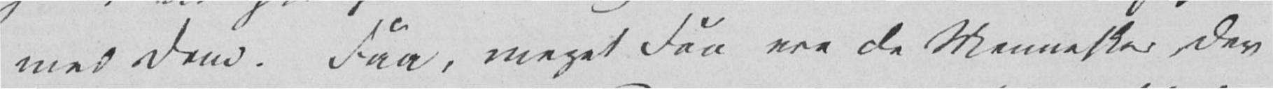med Dem. Faa, meget Faa ere de Mennesker, der
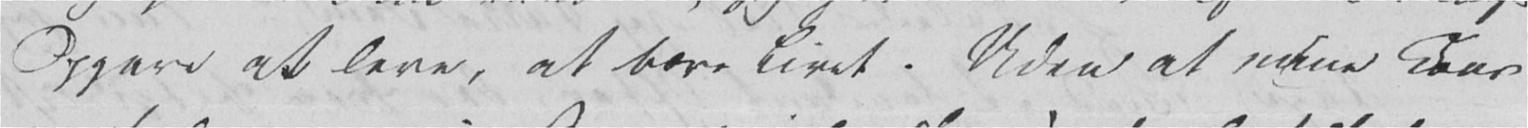Opgave at leve, at bære Livet. Uden at mine Kaar
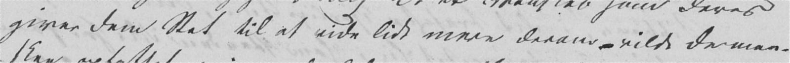giver Dem Ret til at vide lidt mere derom – vilde De maa-
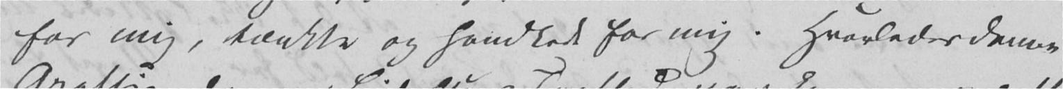for mig, tænkte og handlede for mig. Hvorledes denne
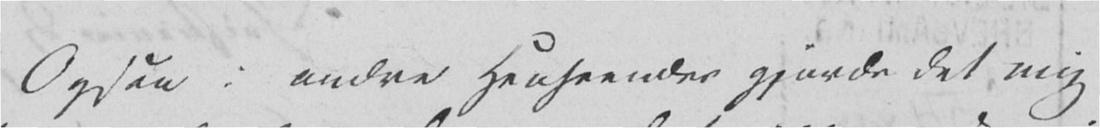Ogsaa i andre Henseender gjorde det mig
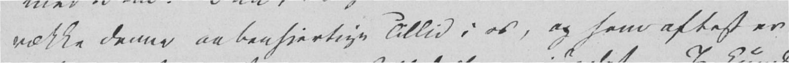vække denne aabenhjertige Tillid i os, og som oftest er
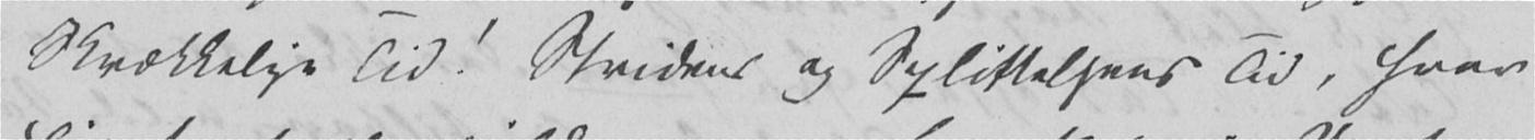Skrækkelige Tid! Stridens og Splittelsens Tid, hvor
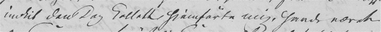indtil den Dag Collett hjemførte mig, havde været
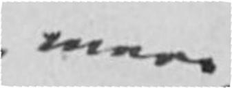mere
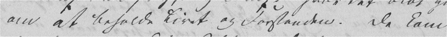om at beholde Livet og Forstanden. De kom
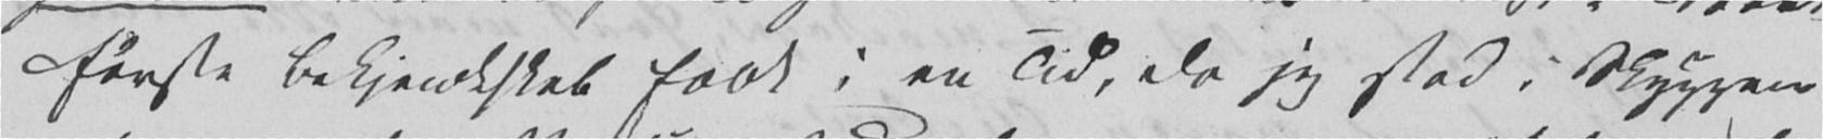første Bekjendtskab faldt i en Tid, da jeg stod i Skyggen
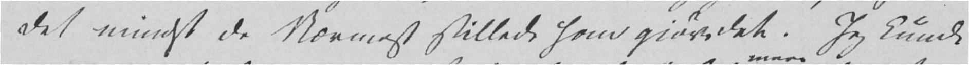det mindst de Nærmest stillede som gjøre det. Jeg kunde
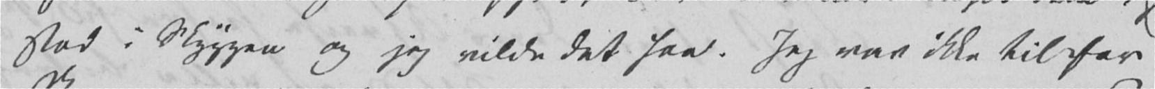stod i Skyggen og jeg vilde det saa. Jeg var ikke til for
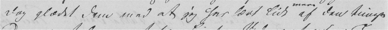dog glædet Dem med at jeg har lært Lidt af den tunge
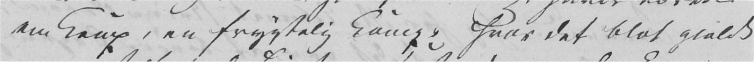en Kamp, en frygtelig Kamp, hvor det blot gjaldt
